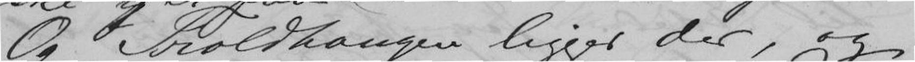Og Troldhaugen ligger der, og
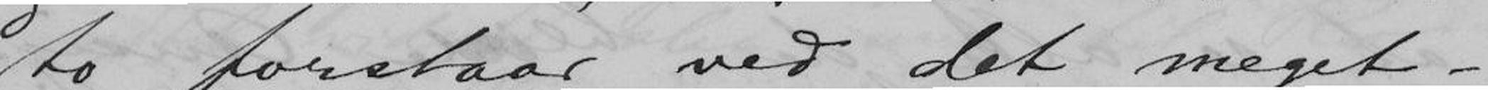to forstaar ved det meget-
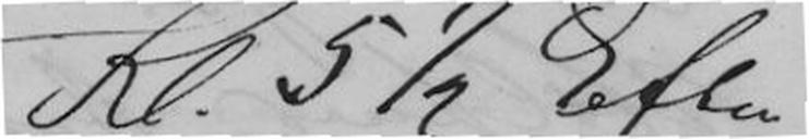Kl. 5 ½ Efter
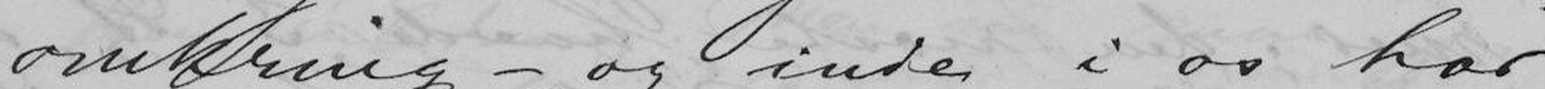omkring - og inde i os har
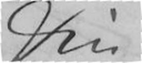Din
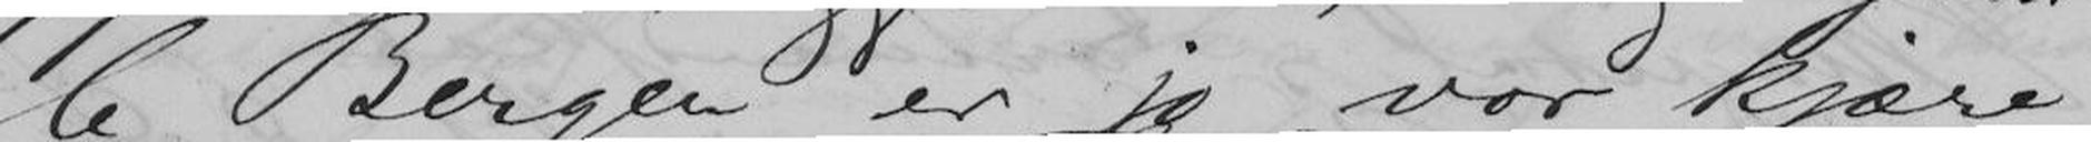le Bergen er jo vor kjære
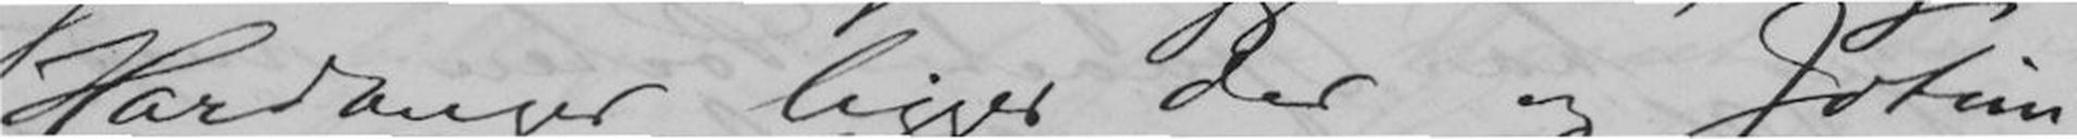Hardanger ligger der og Jotun-
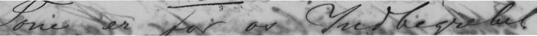Tone er for os Indbegrebet
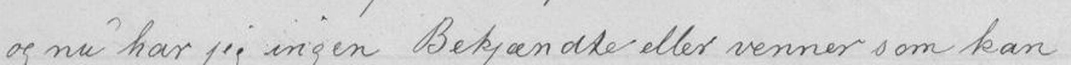og nu har jeg ingen Bekjændte eller venner som kan
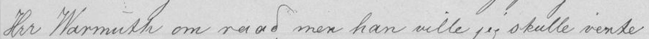Hrr Warmuth om raad, men han ville jeg skulle vente
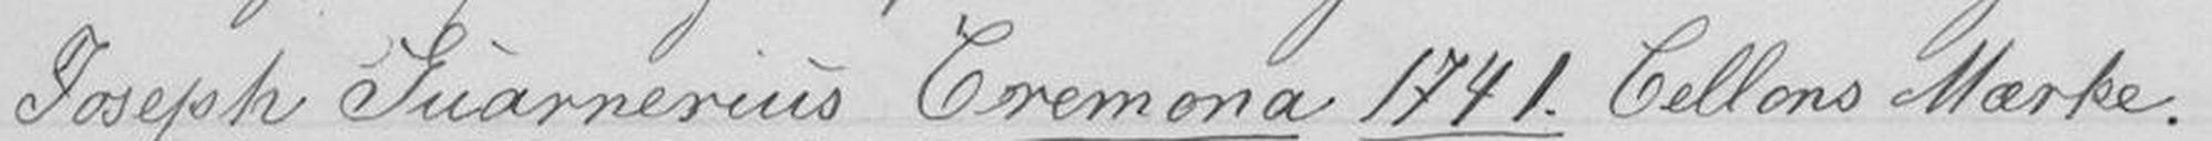Joseph Guarnerius Cremona 1741. Cellons Mærke
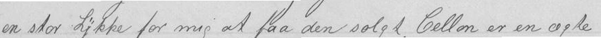en stor Lykke for mig at faa den solgt, Cellon er en ægte
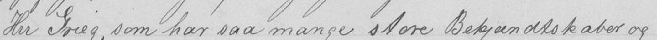Hrr Grieg, som har saa mange store Bekjændtskaber og
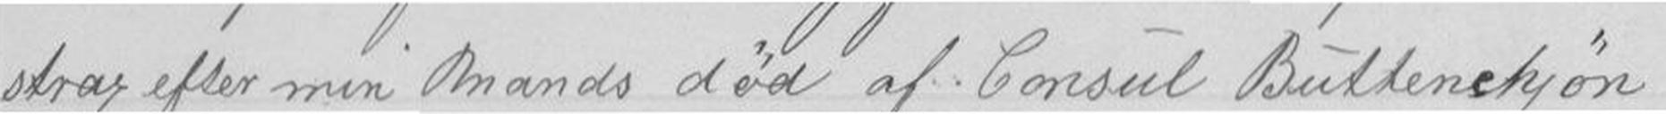strax efter min Mands død af Consul Buttenchjön
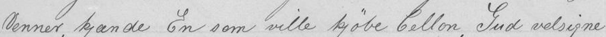Venner, kjænde En som ville kjøbe Cellon, Gud velsigne
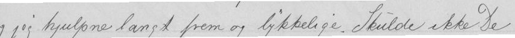og jeg hjulpne langt frem og lykkelige. Skulde ikke De
